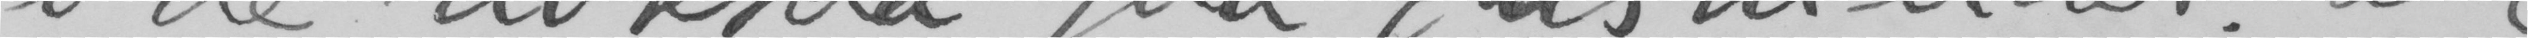i de noksaa faa fristunder. De
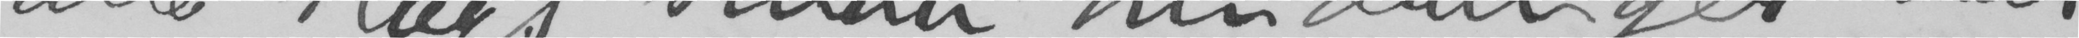alle slags smaa hindringer har
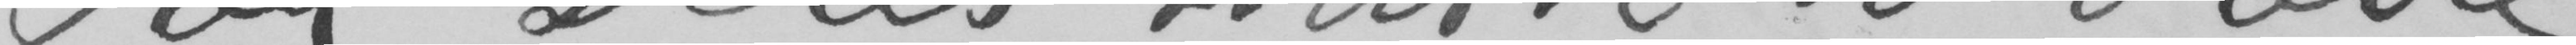For Deres sidste to breve
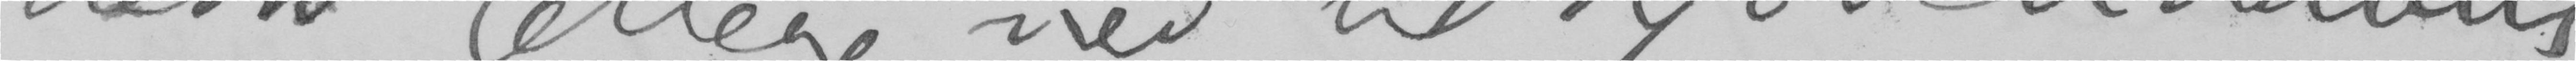desto lettere ned til Kjøbenhavs
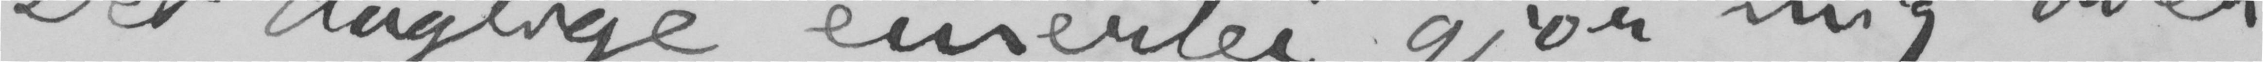Det daglige einerlei gjør mig over-
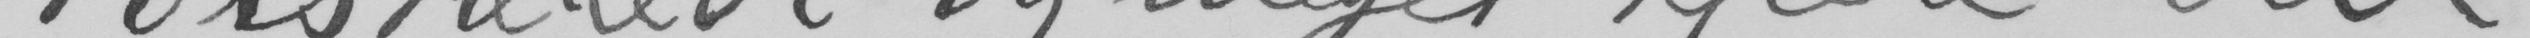Høistærede og meget kjære ven!
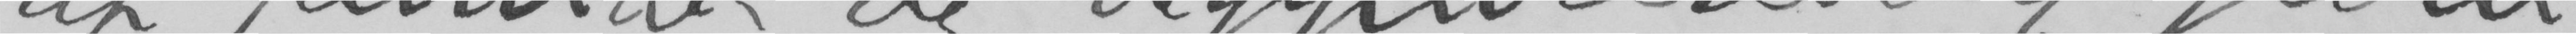af januar og begyndelsen af febru-
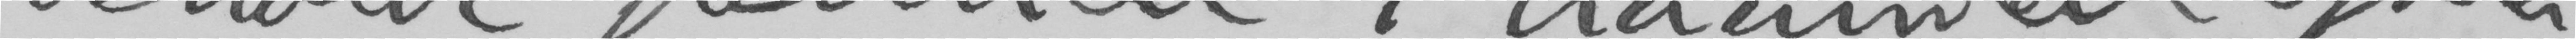beholde pennen i haanden ogsaa
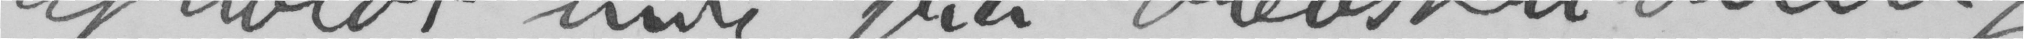afholdt mig fra brevskrivning.
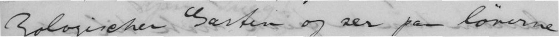Zologischer Garten og ser paa løverne
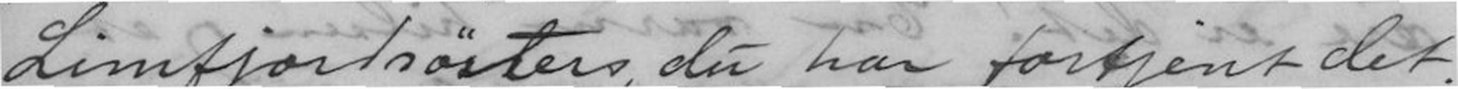Limfjordsøsters, du har fortjent det.
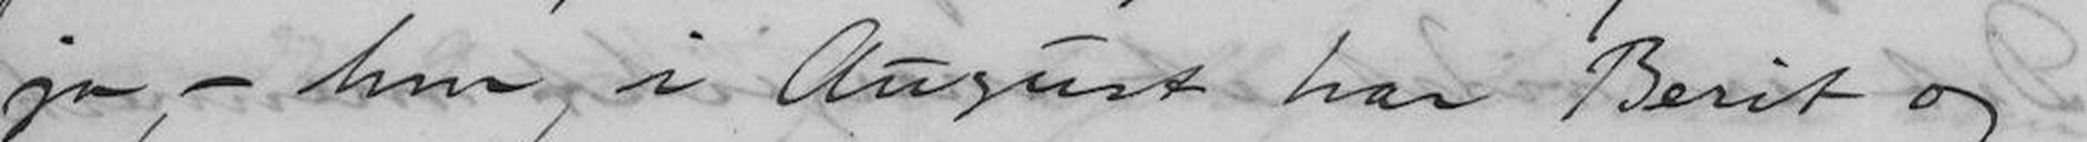ja, - hm, i August har Berit og
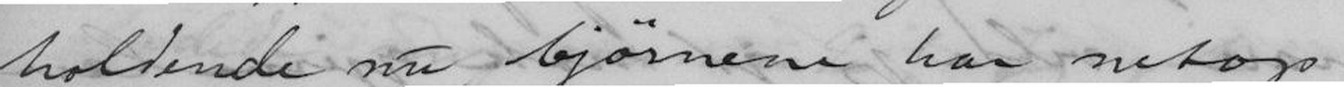holdende nu, bjørnene har netop
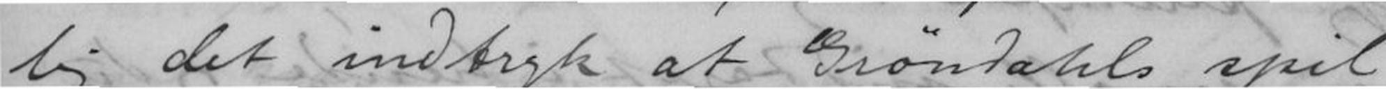lig det indtryk at Grøndahls spil
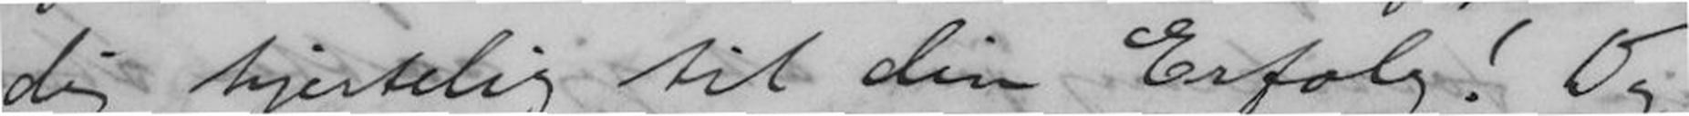dig hjertelig til din Erfolg! Og
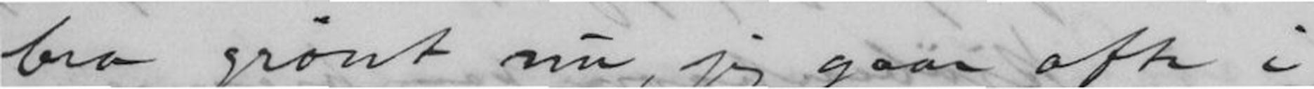bra grønt nu, jeg gaar ofte i
